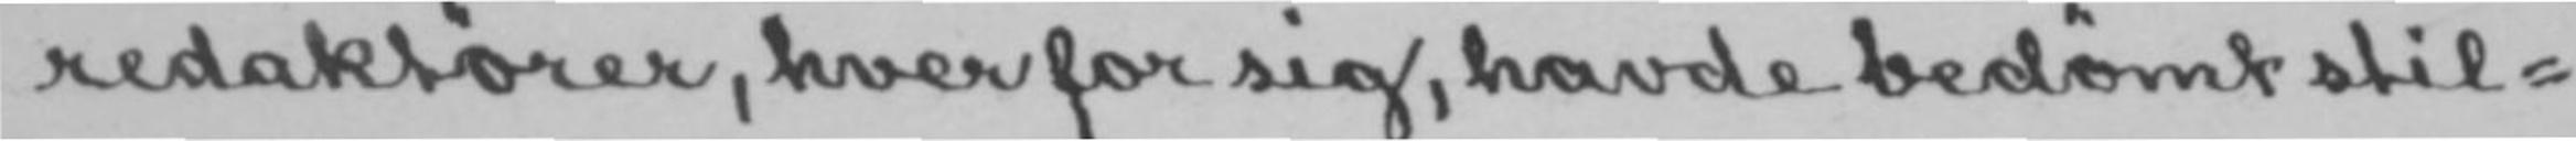redaktører, hver for sig, havde bedømt stil-
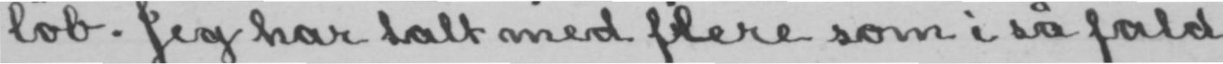løb. Jeg har talt med flere som i så fald
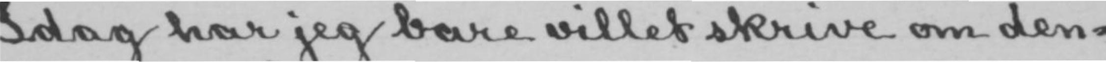Idag har jeg bare villet skrive om den-
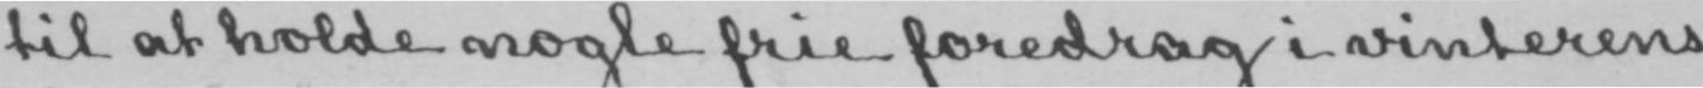til at holde nogle frie foredrag i vinterens
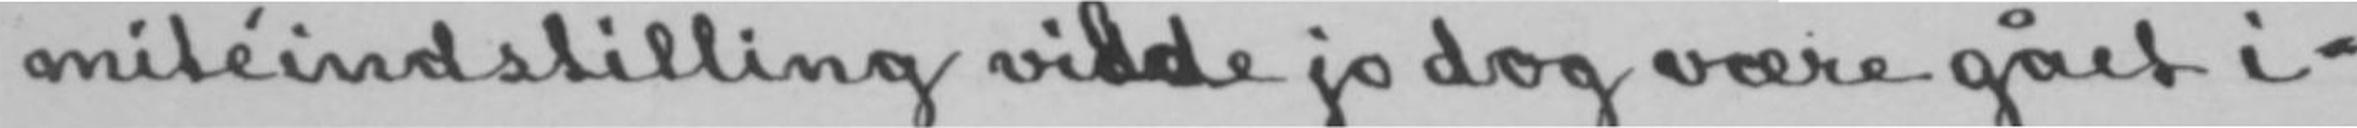mitéindstilling vilde jo dog være gået i-
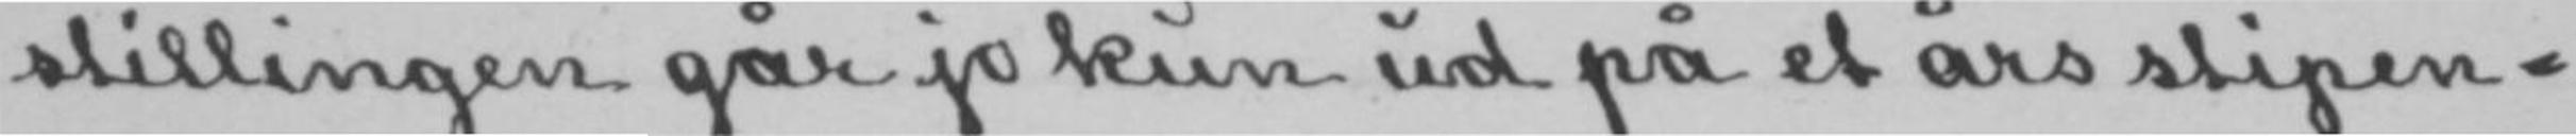stillingen går jo kun ud på et års stipen-
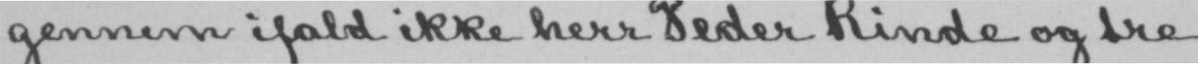gennem ifald ikke herr Peder Rinde og tre
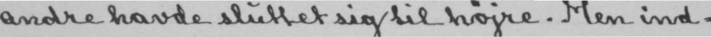andre havde sluttet sig til højre. Men ind-
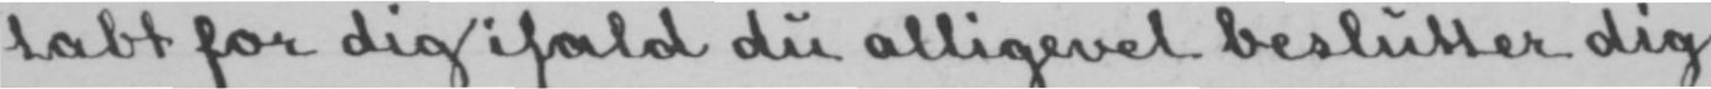tabt for dig ifald du alligevel beslutter dig
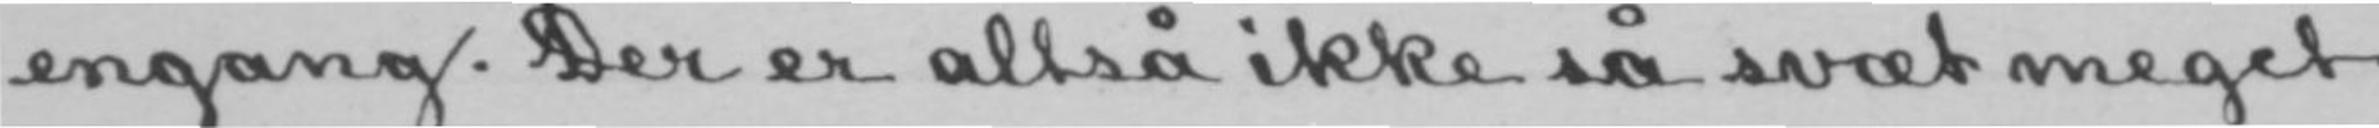engang. Der er altså ikke så svæt meget
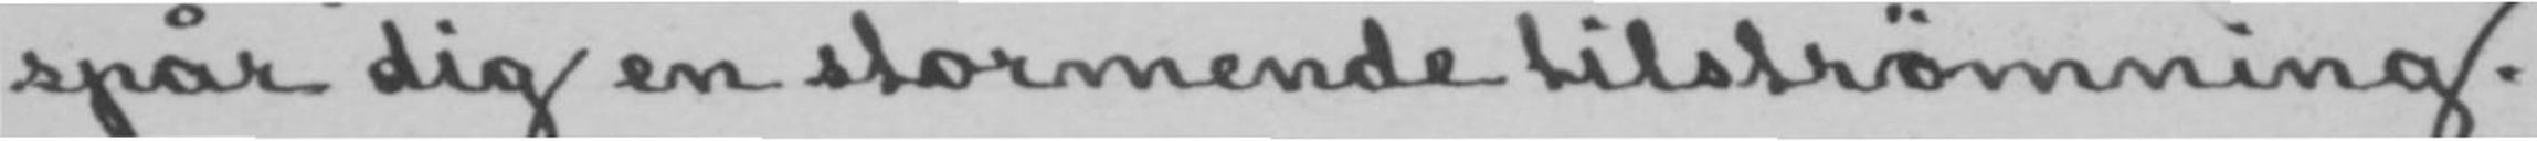spår dig en stormende tilstrømning.
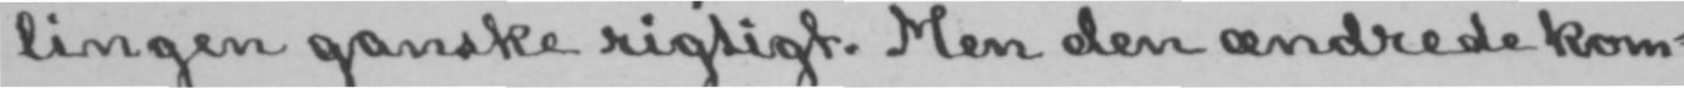lingen ganske rigtigt. Men den ændrede kom-
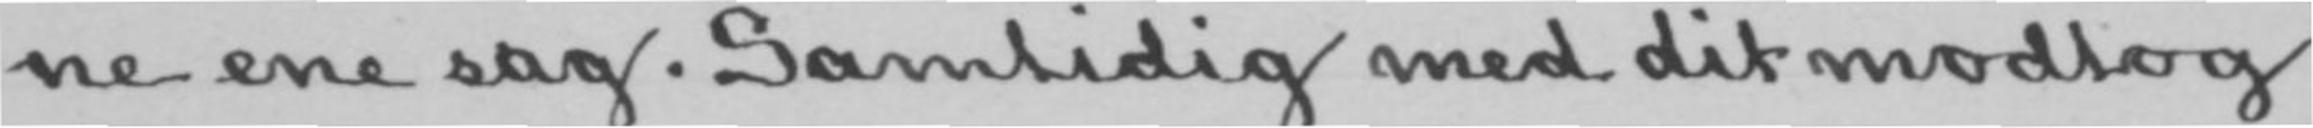ne ene sag. Samtidig med dit modtog
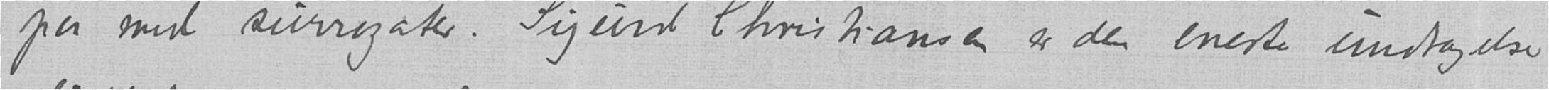paa med surrogater. Sigurd Christiansen er den eneste undtagelse
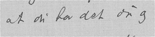at du har det du og.
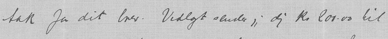tak for dit brev. Vedlagt sender jeg dig kr 200.00 til
